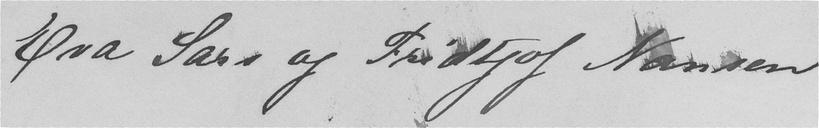Eva Sars og Fridtjof Nansen
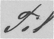Til
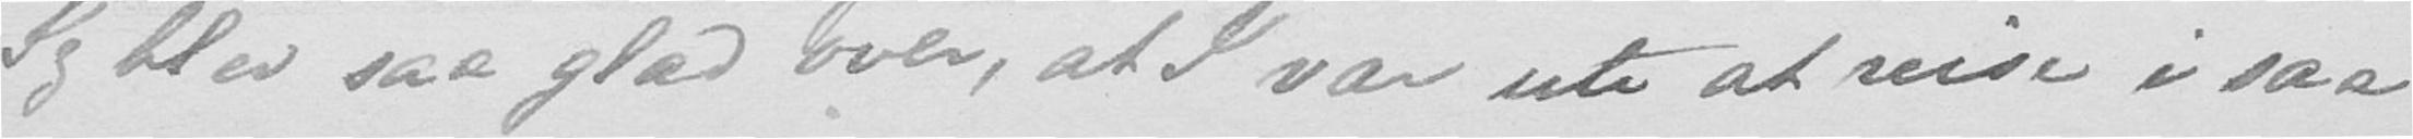Jeg blev saa glad over, at I var ute at reise i saa
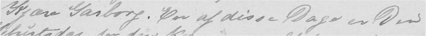Kjære Garborg. En af disse Dage er Din
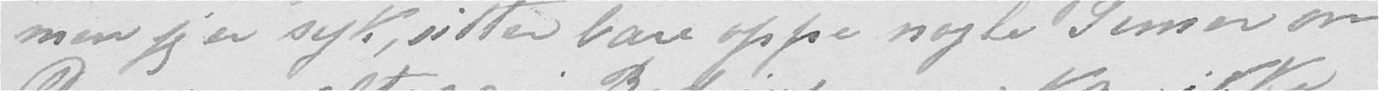men jeg er syk, sitter bare oppe nogle Timer om
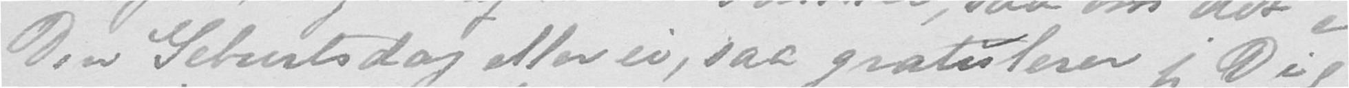Din Geburtsdag eller ei, saa gratulerer jeg Dig
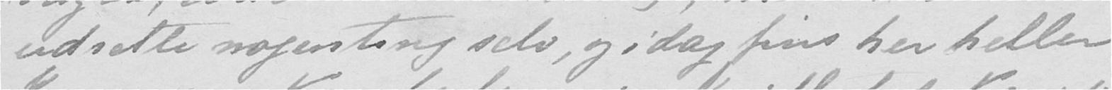udrette nogenting selv, og i dag fins her heller
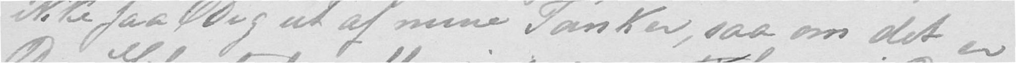ikke faa Dig ut af mine Tanker, saa om det er
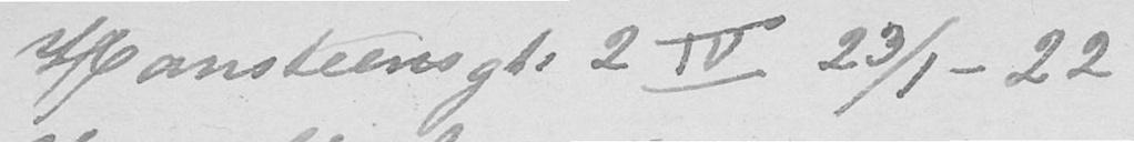Hansteensgt. 2 IV  23/1 - 22
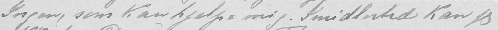Ingen, som kan hjelpe mig. Imidlertid kan jeg
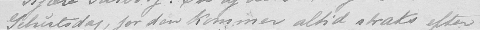Geburtsdag, for den kommer altid straks efter
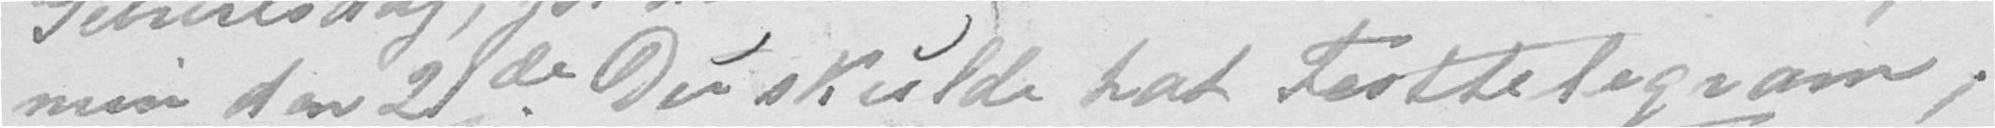min den 21de. Du skulde hat Festtelegram,
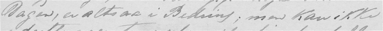Dagen, er altsaa i Bedring; men kan ikke
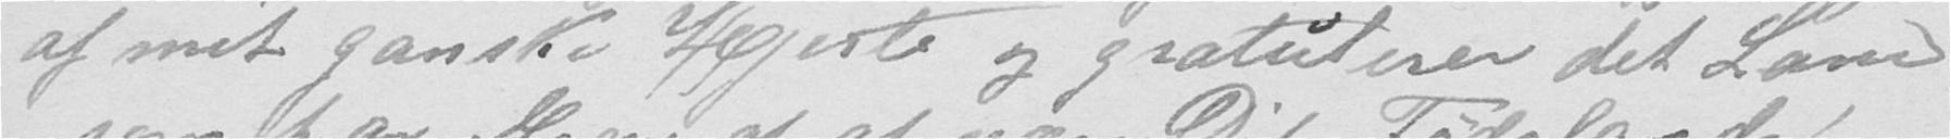af mit ganske Hjerte og gratulerer det Land
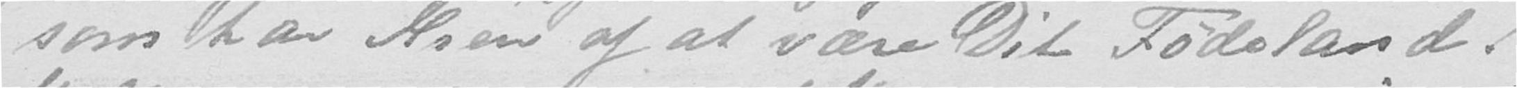som har Æren af at være Dit Fødeland!
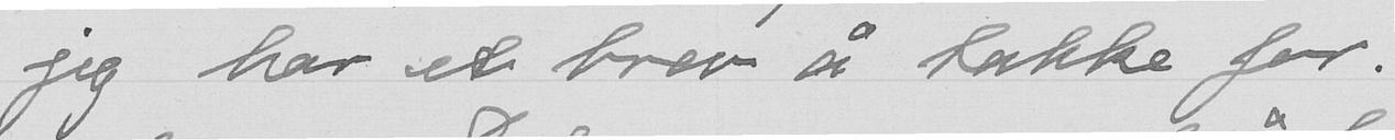jeg har et brev å takke for.
